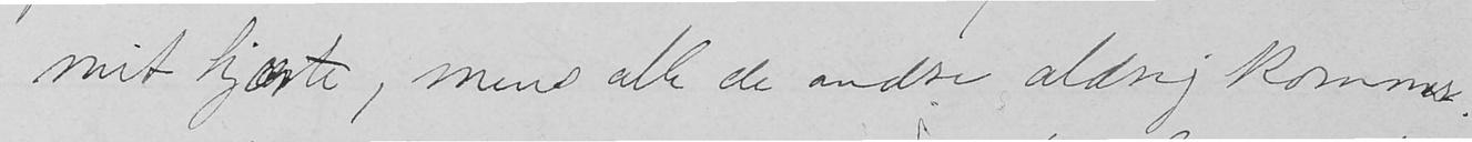mit hjærte, mens alle de andre aldrig kommer
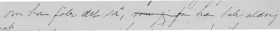om han føler det så, men jeg for han taler aldrig
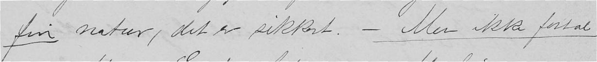fin natur, det er sikkert. - Men ikke fortæl
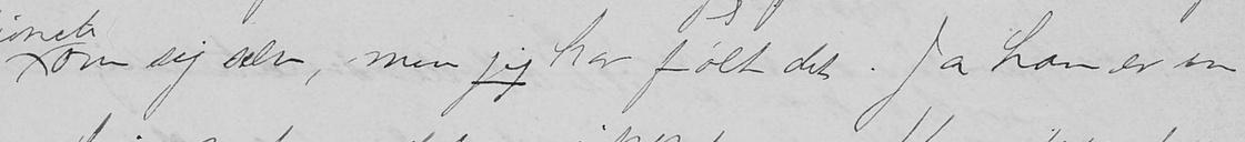om sig selv, men jeg har følt det. Ja han er en
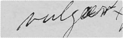vulgær-
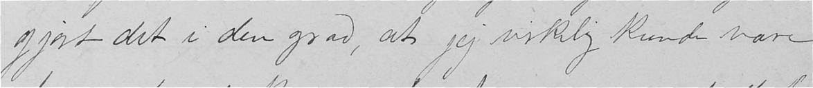gjort det i den grad, at jeg virkelig kunde være
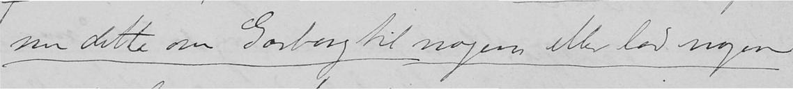nu dette om Garborg til nogen eller lad nogen
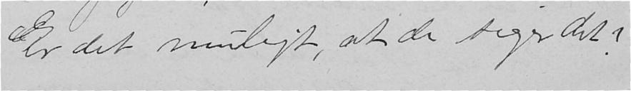Er det muligt, at de siger det?
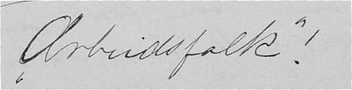"Arbeidsfolk"!
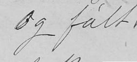og følt
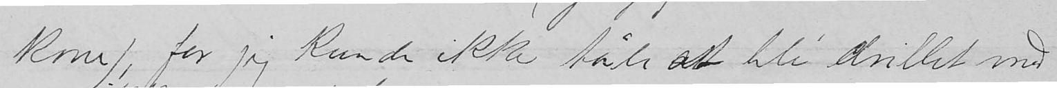kone), for jeg kunde ikke tåle at bli drillet med
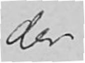der
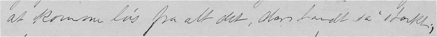at komme løs fra alt det, der bandt så stærkt,
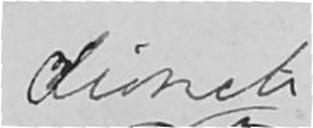direkte
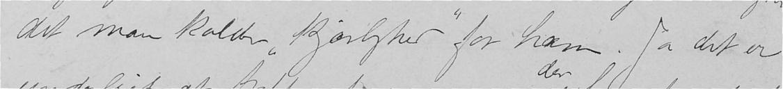det man kalder "kjærlighed" for ham. Ja det er
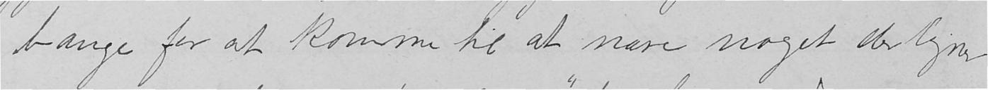bange for at komme til at nære noget der ligner
